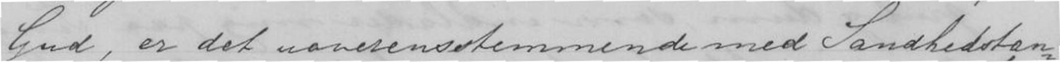Gud, er det uoverenstemmende med Sandhedstan-
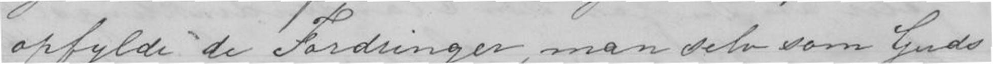opfylde de Fordringer, man selv som Guds
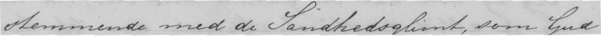stemmende med de Sandhetsglimt, som Gud har
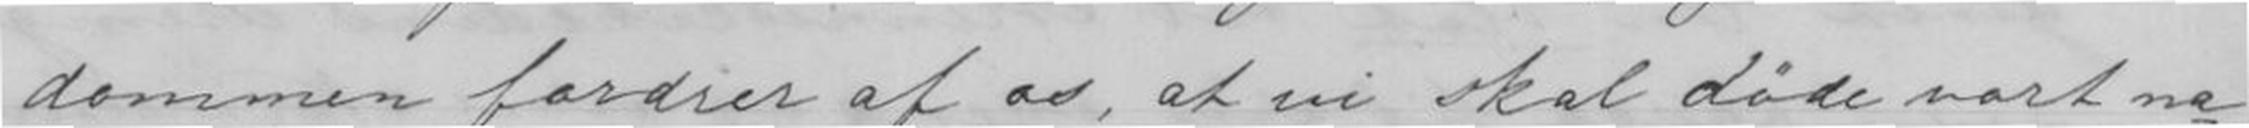dommen fordrer af os, at vi skal døde vort na-
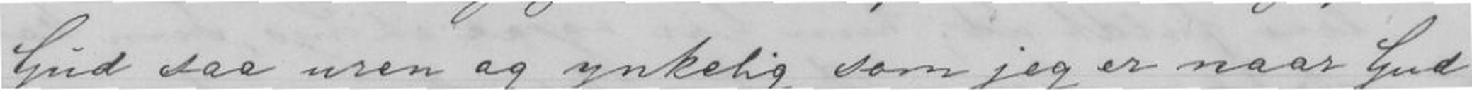Gud saa uren og ynkelig som jeg er naar Gud
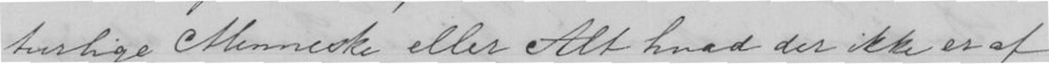turlige Menneske eller Alt hvad der ikke er af
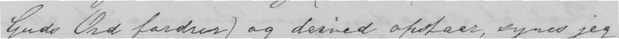Guds Ord fordrer)og derved opstaar, synes jeg,
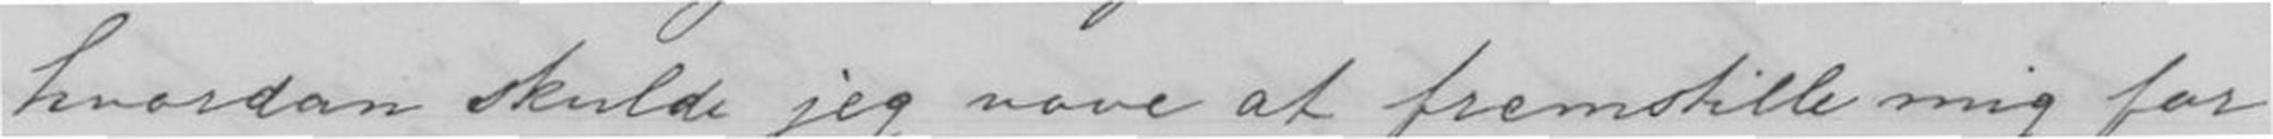hvordan skulde jeg vove at fremstille mig for
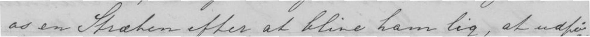os en Stræben efter at blive ham lig, at udfø-
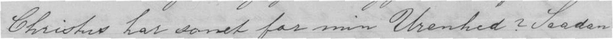Christhus har sonet for min Urenhed? Saadan
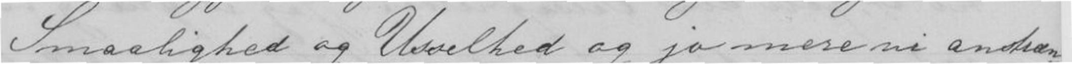Smaalighed og Usselhed og jo mere vi anstræn-
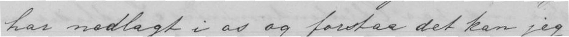nedlagt i os og forstaa det kan jeg
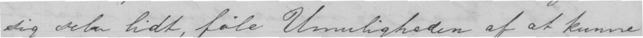sig selv lidt, føle Umuligheten af at kunne
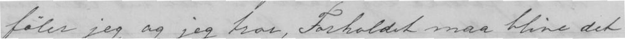føler jeg og jeg tror, Forholdet maa blive det
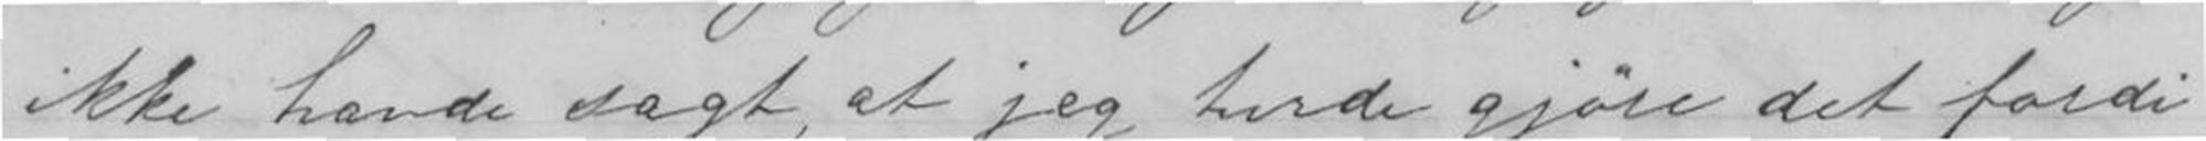ikke havde sagt, at jeg burde gjøre det fordi
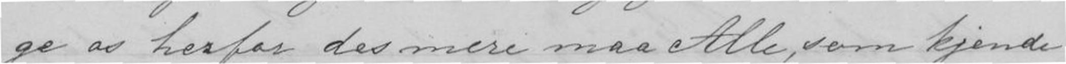ge os herfor des mere maa Alle, som kjende
